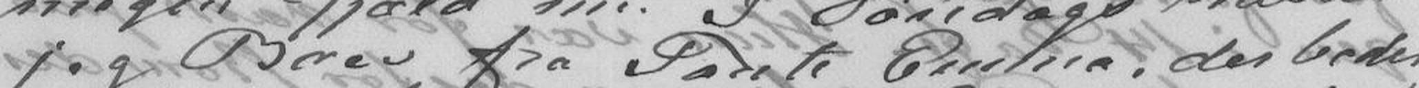jeg Brev fra Tante Emma, der beder
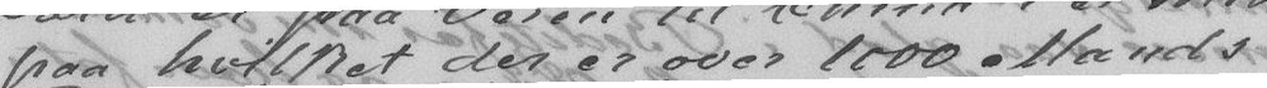paa hvilket der er over 1000 Mands
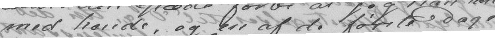med hende, og en af de første Dage
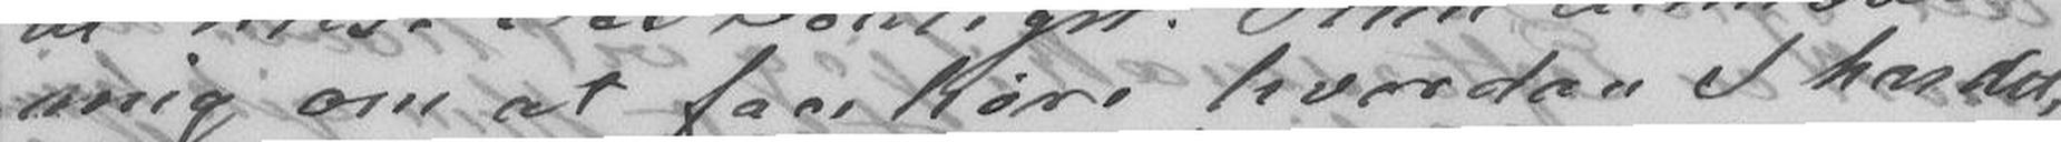mig om at faae høre hvordan I har det,
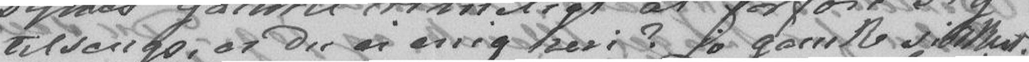tilsengs, at Du ei mig heri? jo ganske sikkert.
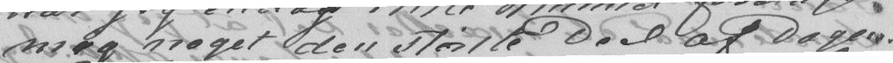mig noget den største Deel af Dagen.
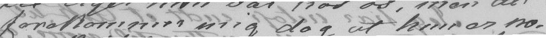forekommer mig dog at hun er no-
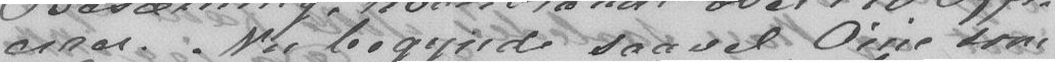cerer. Nu begynde saavel Øiene som
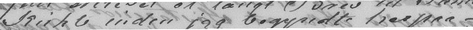Kühle inden jeg begyndte herpaa -
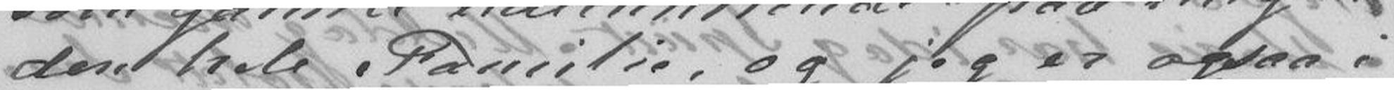den hele Familie, og jeg er ogsaa i
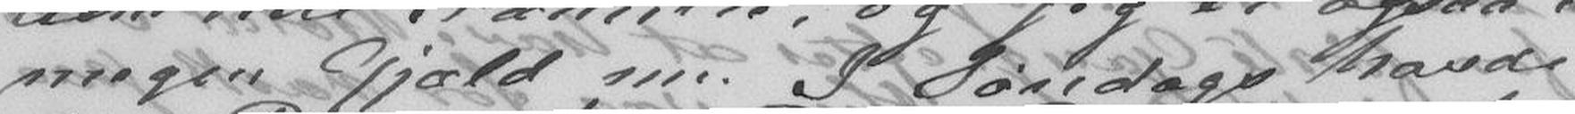megen Gjæld nu. I Søndags havde
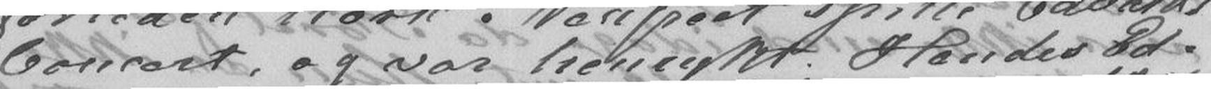Concert, og var henrykt. Hendes Ed-
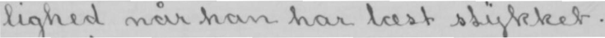lighed når han har læst stykket.
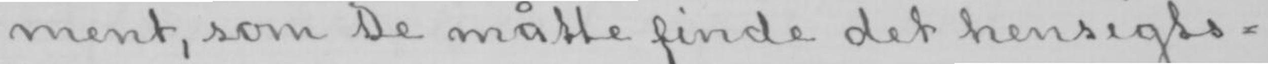ment, som De måtte finde det hensigts-
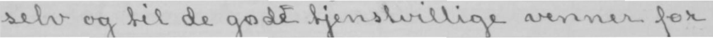selv og til de gode tjenstvillige venner for
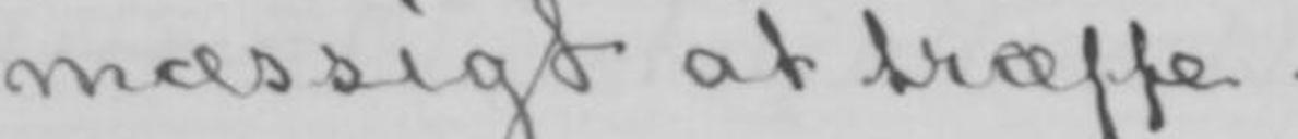mæssigt at træffe.
